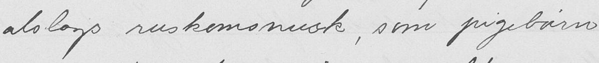alslags ruskomsnusk, som pigebørn
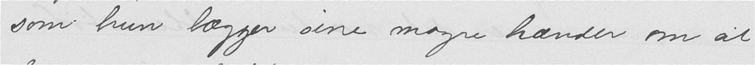som hun lægger sine magre hænder om et
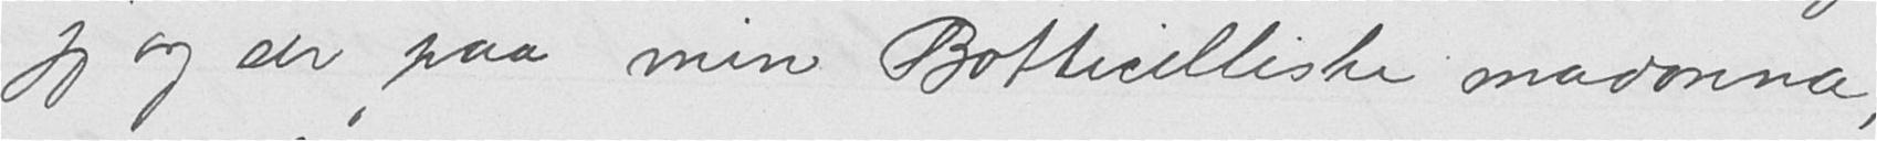jeg og ser paa min Botticelliske madonna,
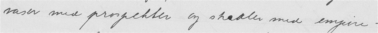vaser med prospekter og skaaler med empire-
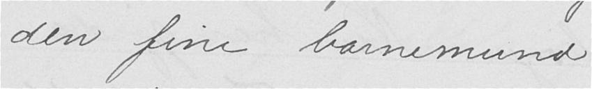den fine barnemund.
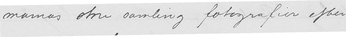mamas store samling fotografier efter
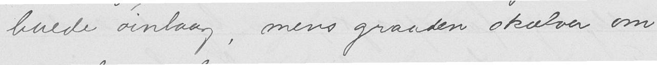buede øinlaag, mens graaden skælver om
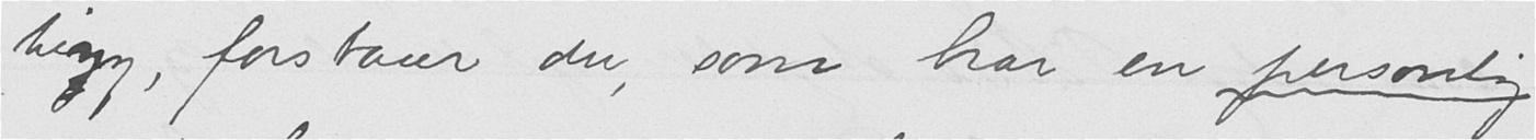ting, forstaar du, som har en personlig
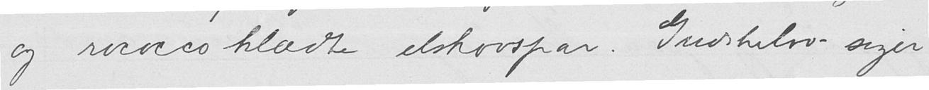og rococcoklædte elskovspar. Gudskelov siger
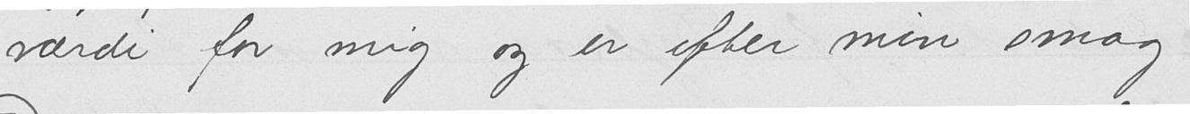værdi for mig og er efter min smag.
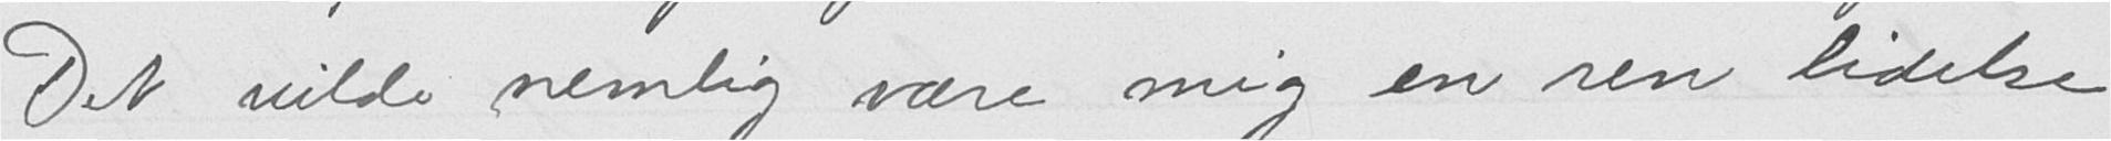Det vilde nemlig være mig en ren lidelse
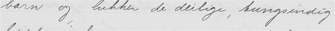barn og lukker de deilige, tungsindig
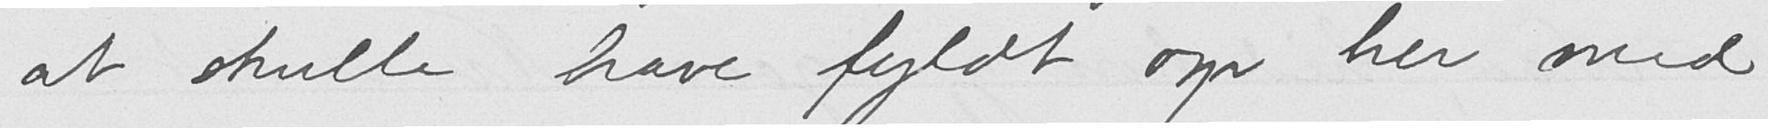at skulle have fyldt op her med
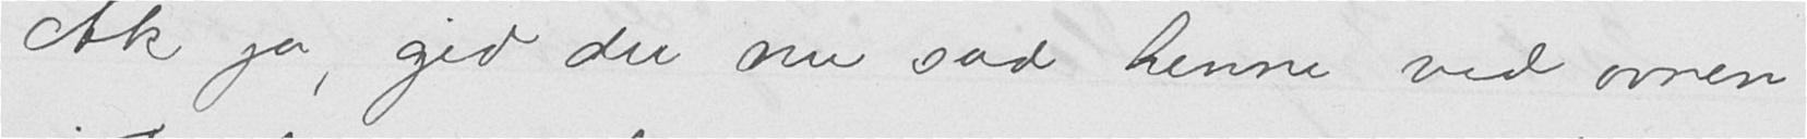Ak ja, gid du nu sad henne ved ovnen
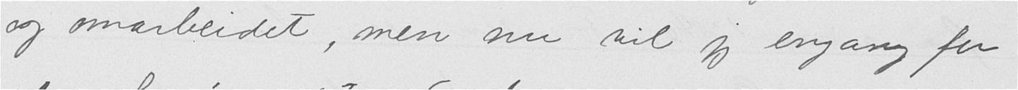og omarbeidet, men nu vil jeg engang for
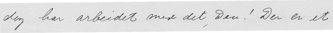dog har arbeidet med det, Dea! Der er et
